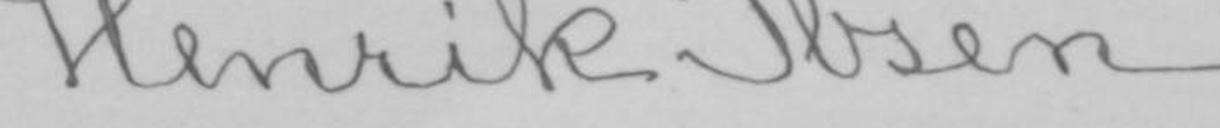Henrik Ibsen.
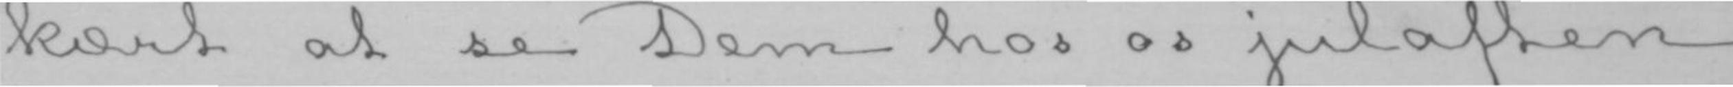kært at se Dem hos os julaften
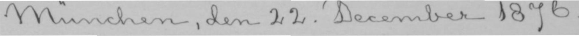München, den 22. December 1876.
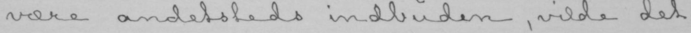være andetsteds indbuden, vilde det
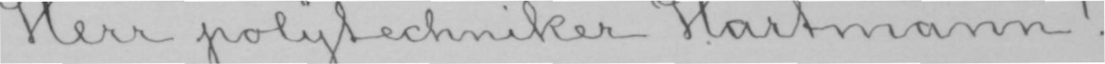Herr polytechniker Hartmann!
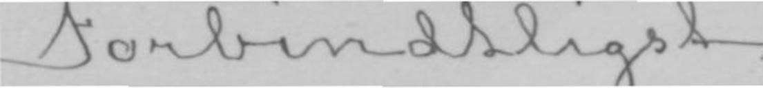Forbindtligst
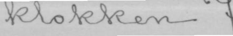klokken 7.-
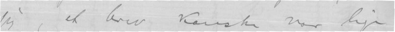jeg, et brev kanske var lige
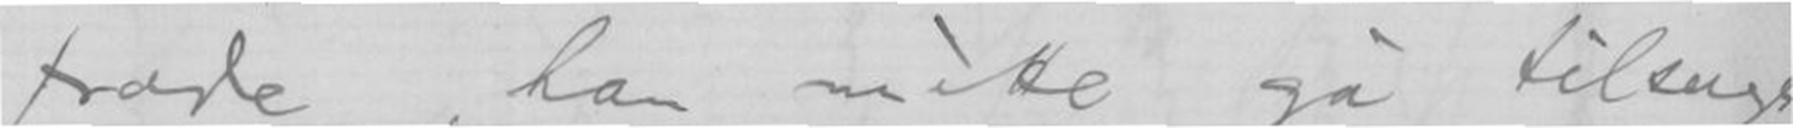trode, han måtte gå tilsengs
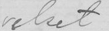velset.
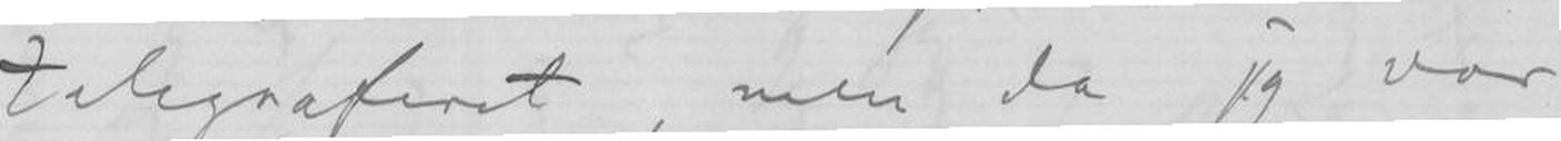telegraferet, men da jeg var
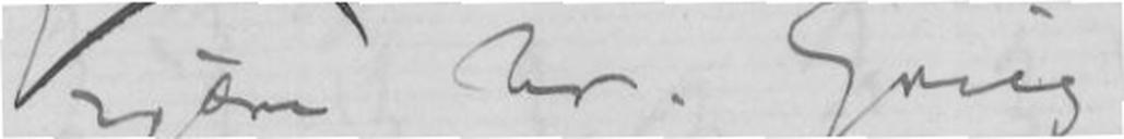Kjære hr. Grieg
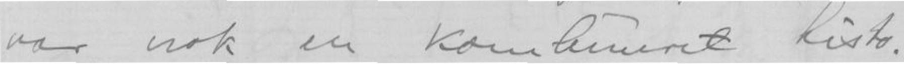var nok en Kombineret histo-
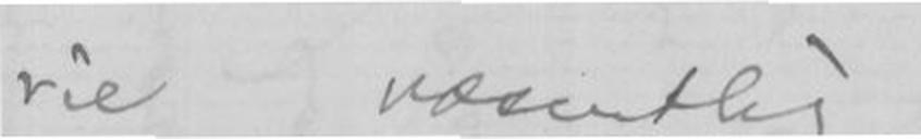rie, væsentlig
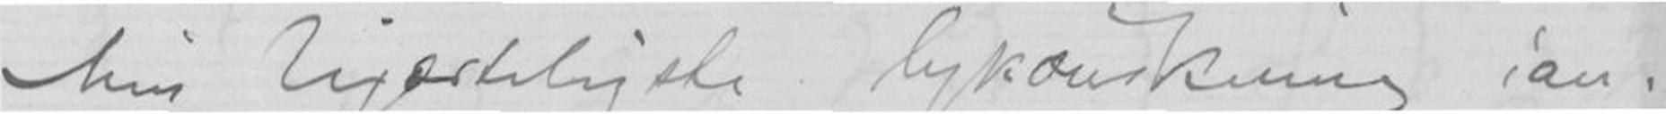Min hjærteligste lykønskning ian-
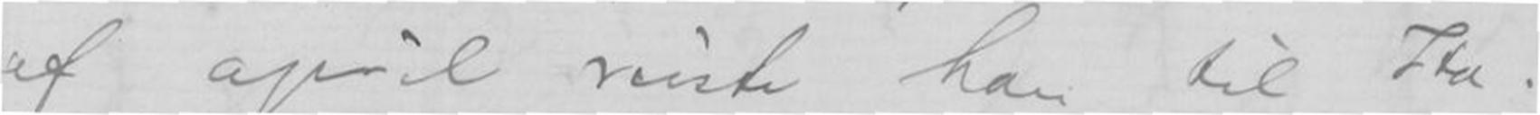af april reiste han til Ita-
